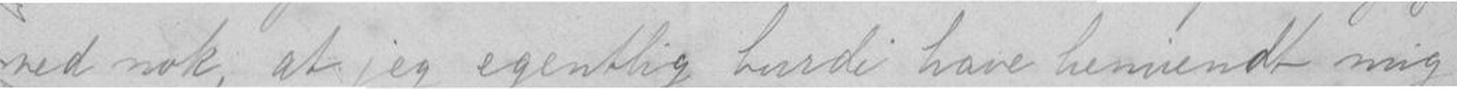ved nok, at jeg egentlig burde have henvendt mig
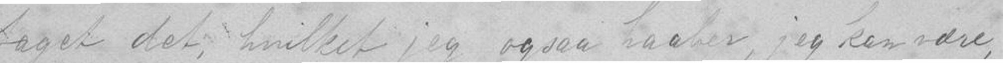taget det, hvilket jeg ogsaa haaber, jeg kan være,
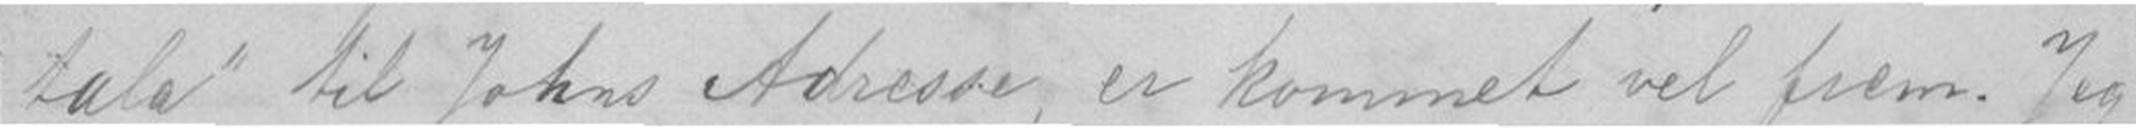taler til Johns Adresse, er kommet vel frem. Jeg
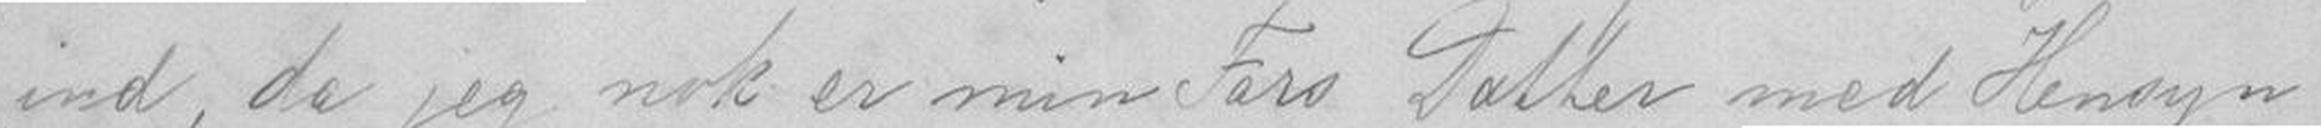ind, da jeg nok er min Fars Datter med Hensyn
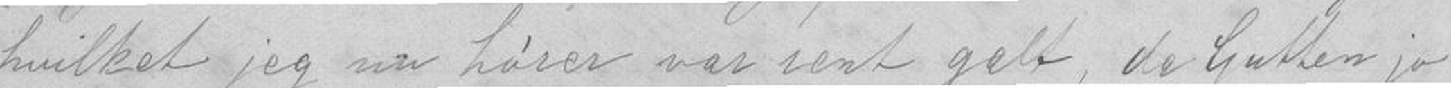hvilket jeg nær hører var rent galt, de Gutten jo
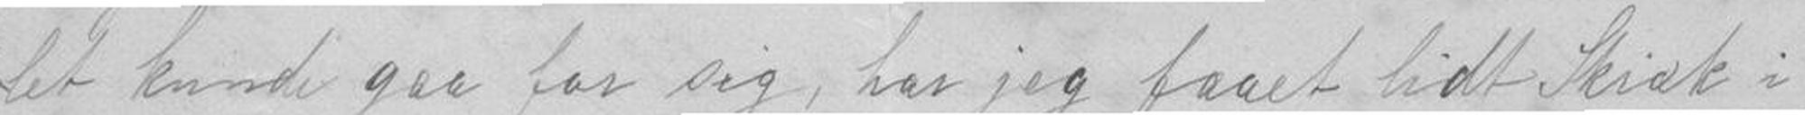let kunde gaa for sig, har jeg faaet lidt Skræk i
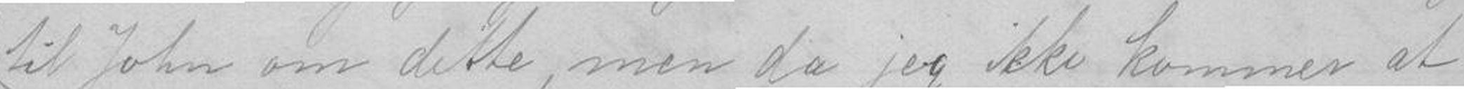til John om dette, men da jeg ikke kommer at
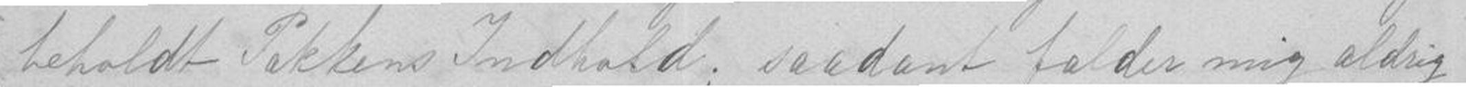beholdt Pakkens Indhold; saadant falder mig aldri
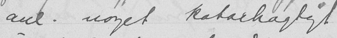anl. noget katarhagtigt
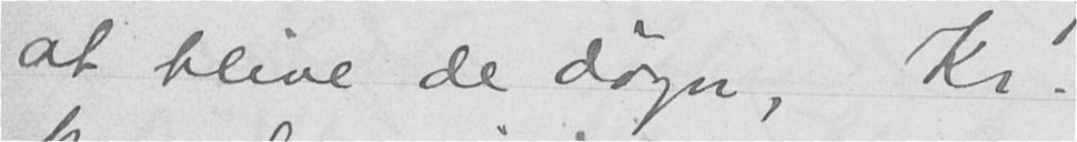at blive de døgn, Kr.
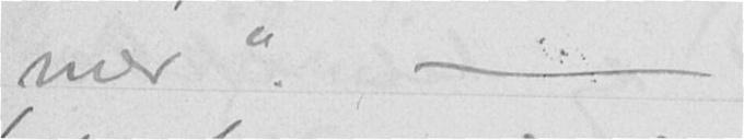mer". -
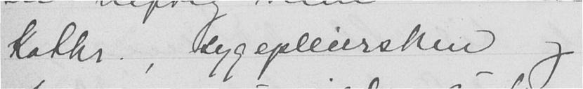Kathr., sygepleiersken og
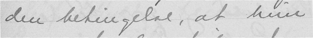den betingelse, at hun
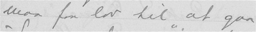maa faa lov til "at gaa
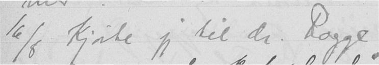16/8 Kjørte jeg til dr. Rogge
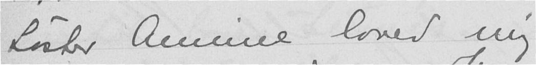Søster Amine loved mig
